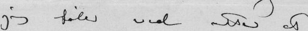jeg føler ved atter at
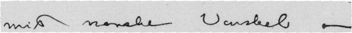mit norske Vanskab -
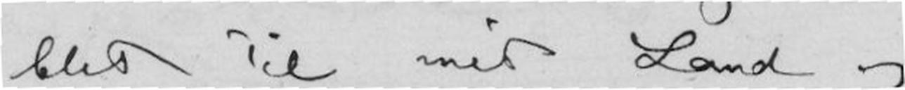blot til mit Land og
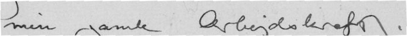min gamle Arbejdskraft.
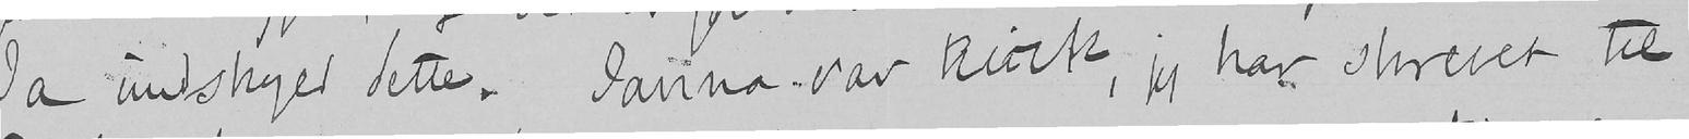Ja undskyld dette. Janna var kvik, jeg har skrevet til
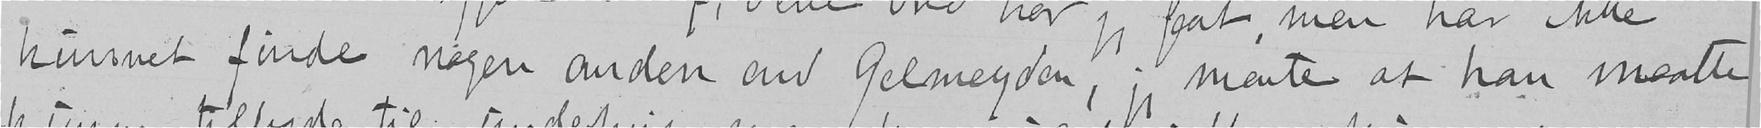kunnet finde nogen anden end Gelmeyden, jeg mente at han maatte
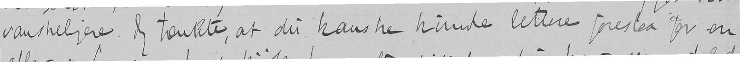vanskeligere. Jeg tænkte, at du kanske kunde lettere foreslaa for en
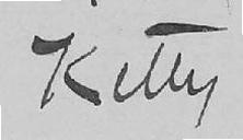Kitty
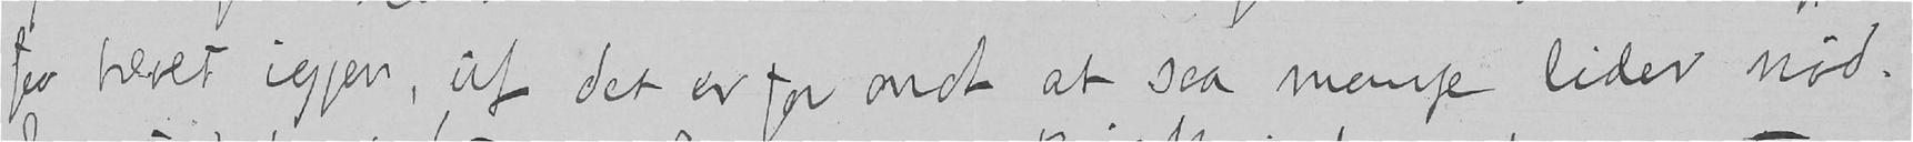faa brevet igjen, uf det er for ondt at saa mange lider nød.
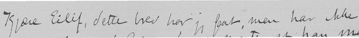Kjære Eilif, dette brev har jeg faat, men har ikke
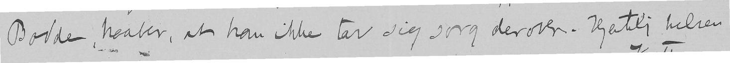Bodde, haaber, at han ikke tar sig sorg derover. Hjertelig hilsen
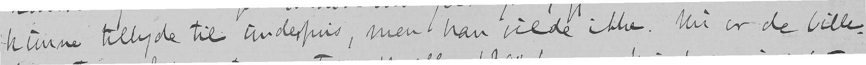kunne tilbyde til underpris, men han vilde ikke. Nu er de bille-
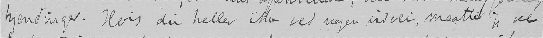kjendinger. Hvis du heller ikke ved nogen udvei, maatte jeg vel
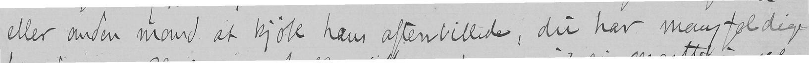eller anden mand at kjøbe hans aftenbillede, du har mangfoldige
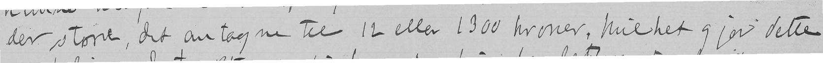der større, det antagne til 12 eller 1300 kroner, hvilket gjør dette
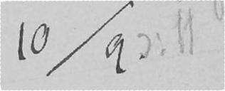10/9
